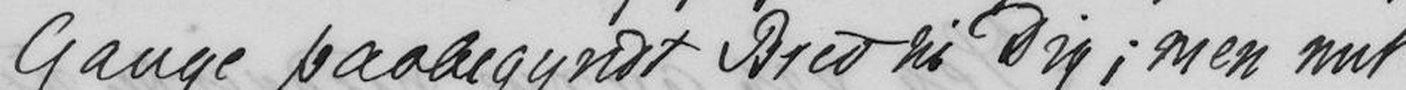Gange paabegyndt Brev til Dig; men mit
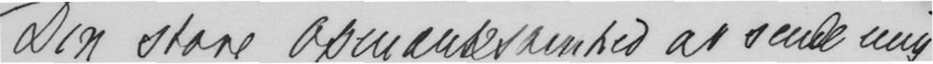Din store Opmærksomhed at sende mig
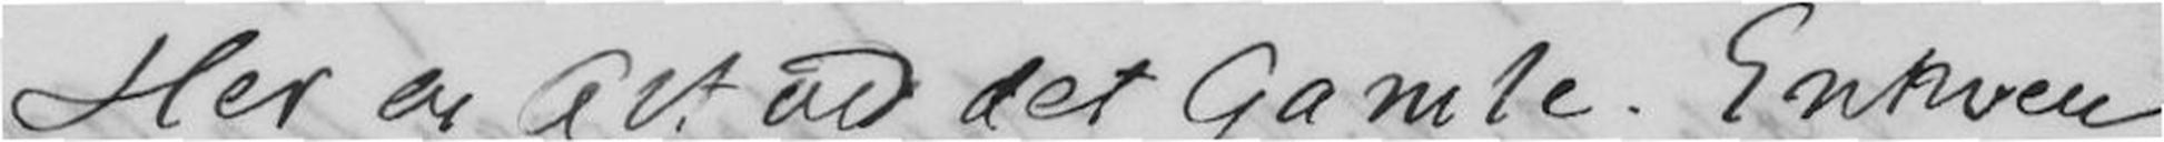Her er alt ved det gamle. Enhver
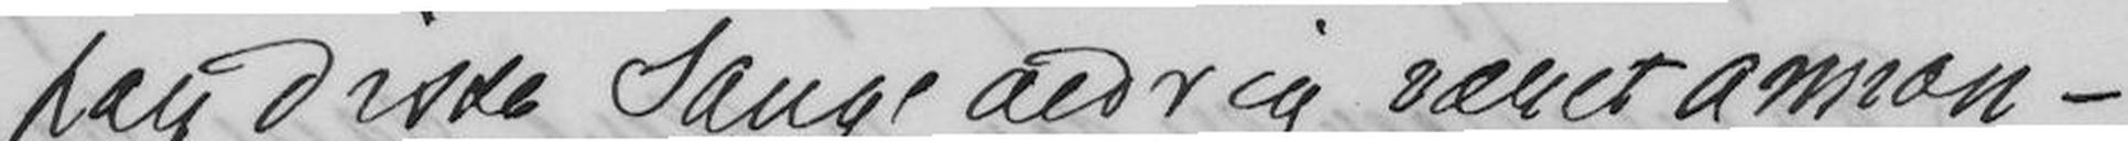har disse Sange aldrig været annon-
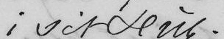i sit Hus.
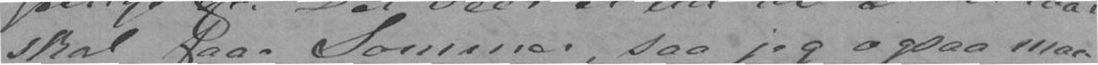skal faae Sommer, saa jeg ogsaa maa
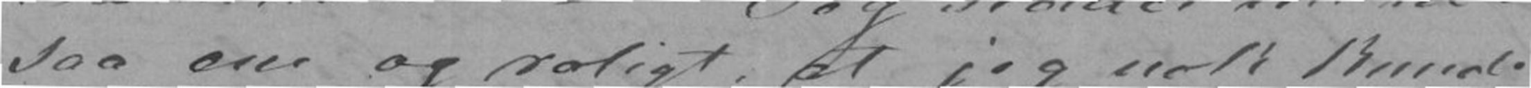saa ene og roligt, at jeg nok kunde
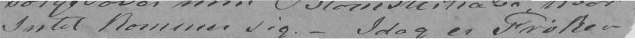Intet kommer sig. - Idag er Frøken
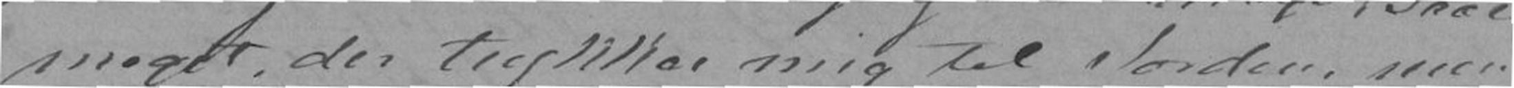meget der trykker mig til Jorden, men
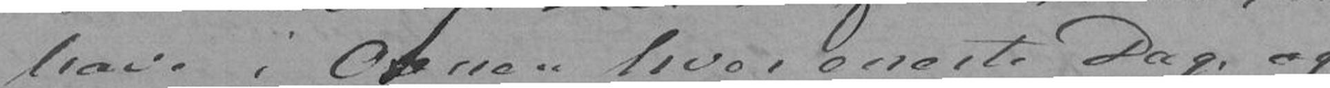have i Ovnen hver eneste Dag, og
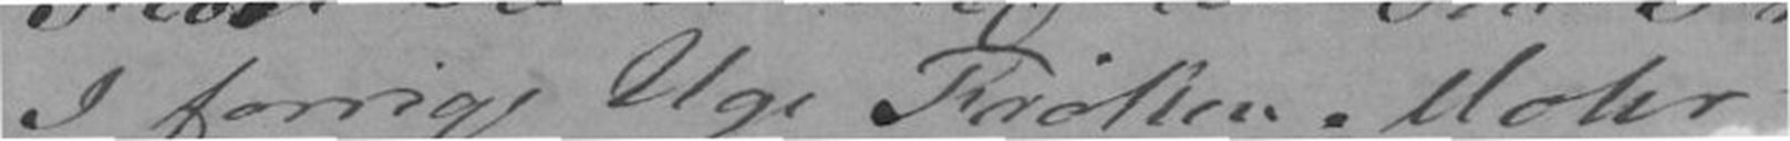I forrige Uge Frøken Mohr
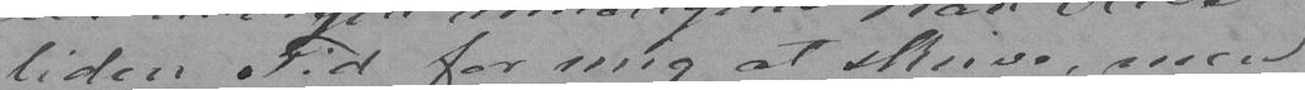liden Tid for mig at skrive, men
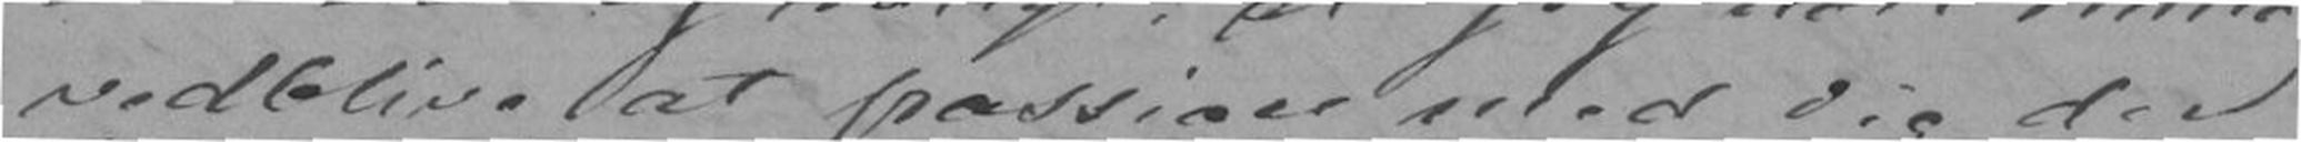vedblive, at passiare med Dig den
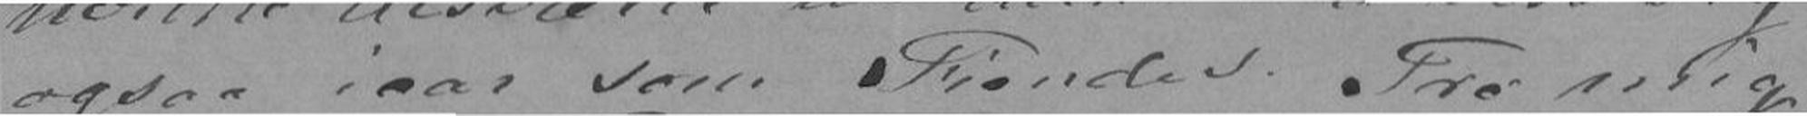ogsaa iaar som Fiender. Tro mig
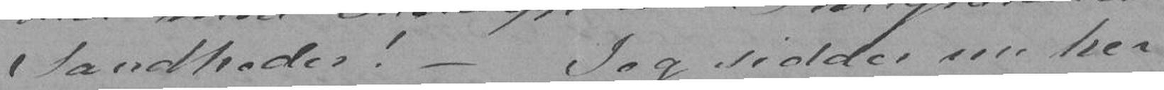Sandheder! - Jeg sidder nu her
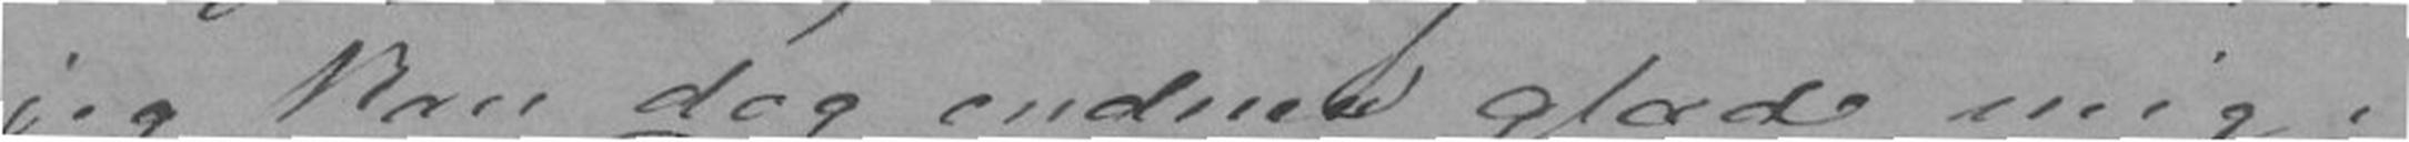jeg kan dog endnu glæde mig i
